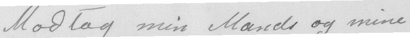Modtag min Mands og mine
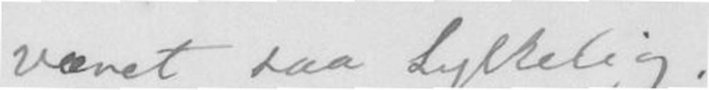været saa Lykkelig
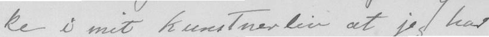ke i mit kunstnerliv at jeg har
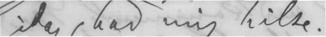idag, bad mig hilse.
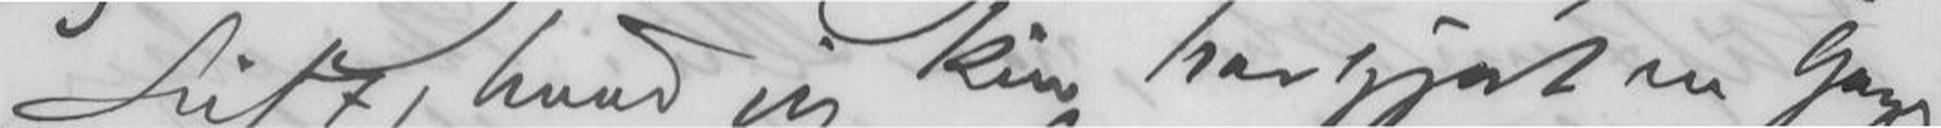Luft, hvad jeg kun har gjort en Gang
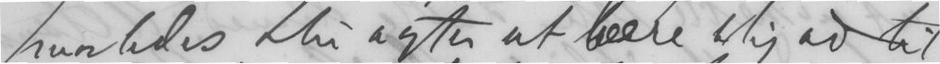hvorledes Du agter at bære Dig ad til
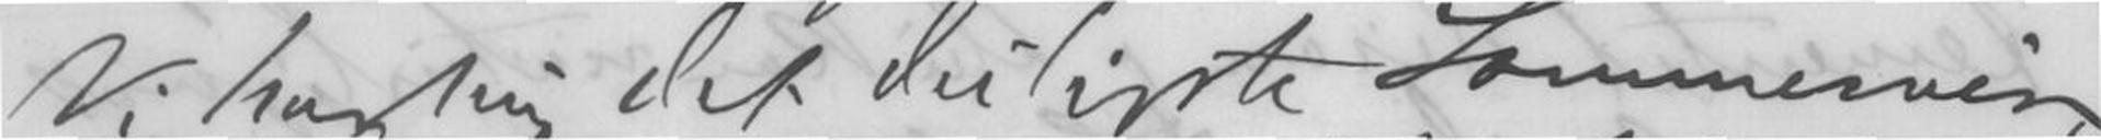Vi har nu det deiligste Sommerveir,
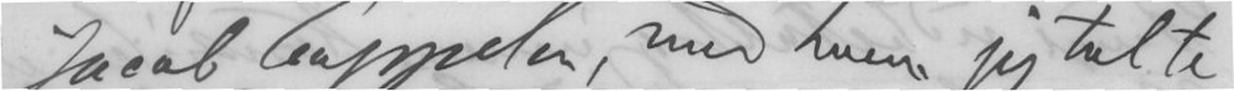Jacob Cappelen, med hvem jeg talte
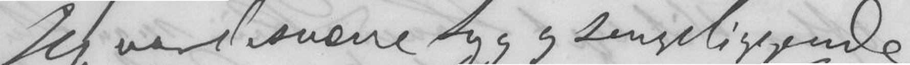Jeg var desværre syg og sengeliggende,
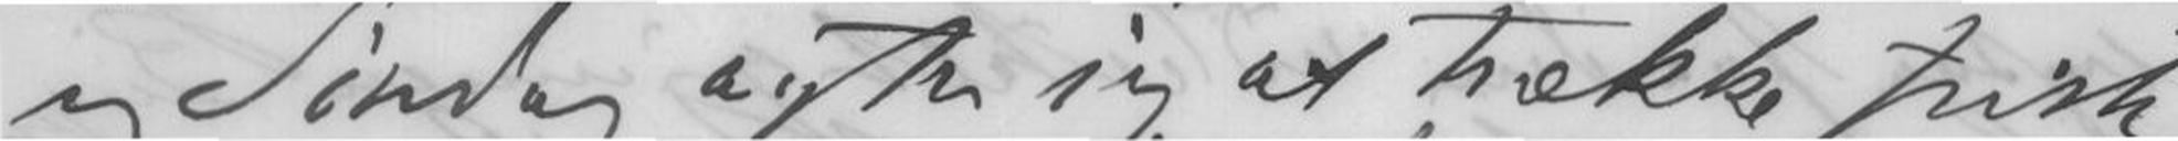og Søndag agter jeg at trække frisk
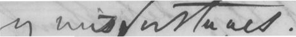og misforstaaes.
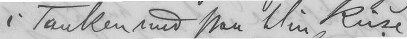i Tanken med paa Din Reise.
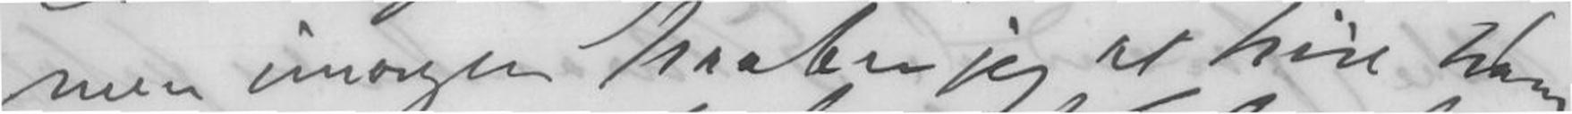men imorgen haaber jeg at høre ham.
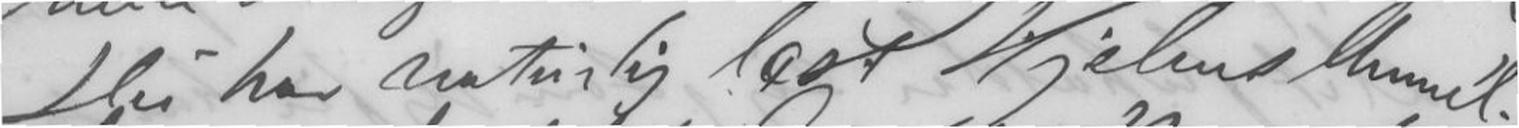Du har naturligvis læst Hjelms Anmel-
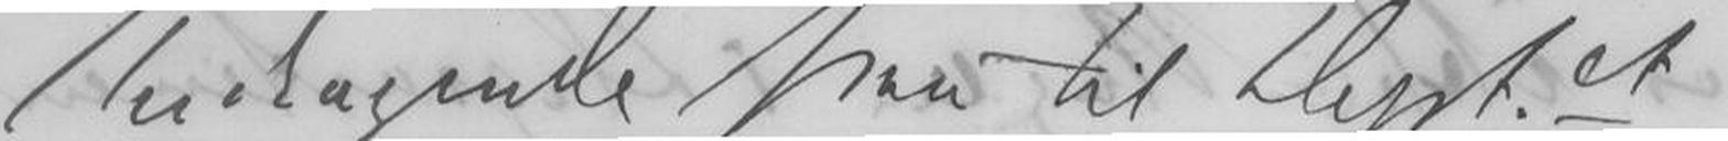Andragnede paa til Dept.et
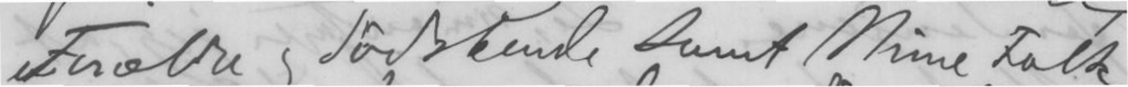Forældre og Søskende samt Mine Folk,
